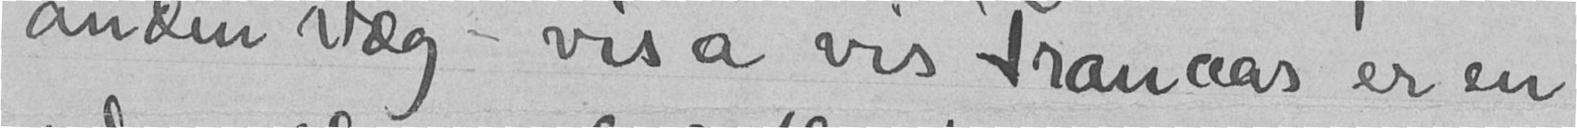anden væg - vis a vis Tranaas er en
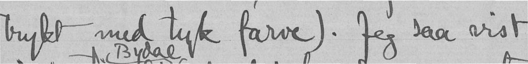trykt med tyk farve). Jeg saa vist
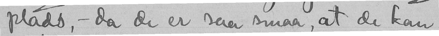plads, - da de er saa smaa, at de kan
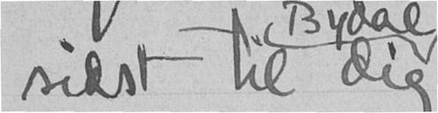sidst til dig
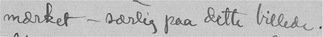mærket - særlig paa dette billede.
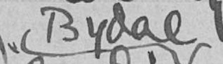Bydal
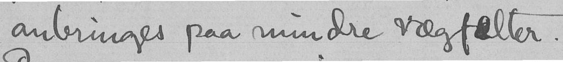anbringes paa mindre vægfælter.
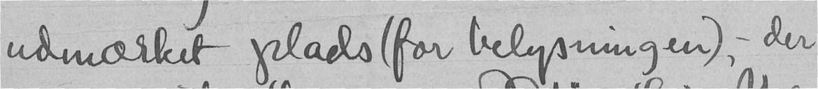udmærket plads (for belysningen) - der
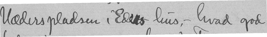Hæderspladsen i Eders hus - hvad god
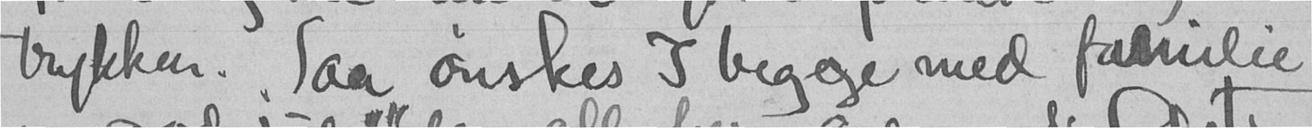trykken. Saa ønskes I begge med familie
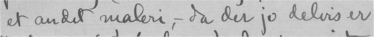et andet malere, - da der jo delvis er
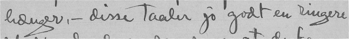hænger, - disse taaler jo godt en ringere
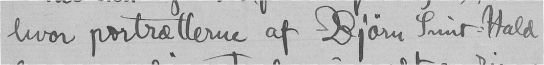hvor portrætterne af Bjørn Smit-Hald
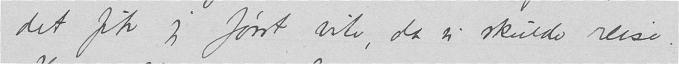det fik jeg først vite, da vi skulde reise.
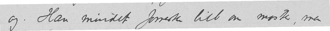og. Han mindet forresten litt om moster, men
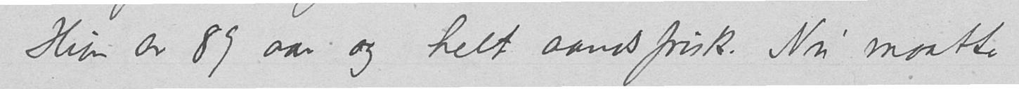Hun er 89 aar og helt aandsfrisk. Nu maatte
